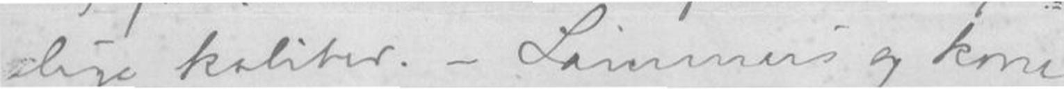lige kaliber.- Lammer's og kone
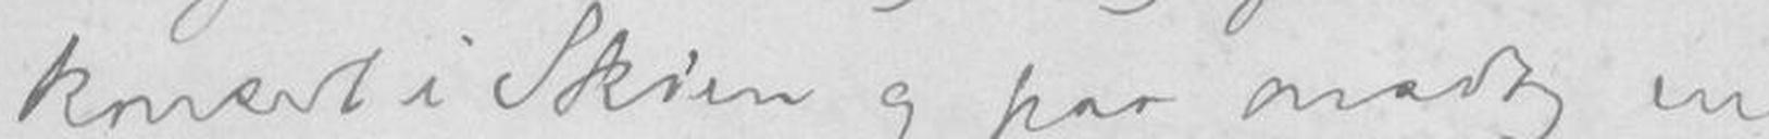koncert i Skien og paa onsdag en
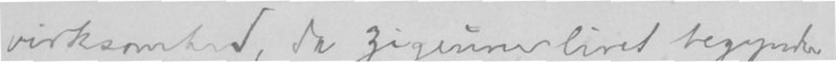virksomhed, da zigeunerlivet begynder
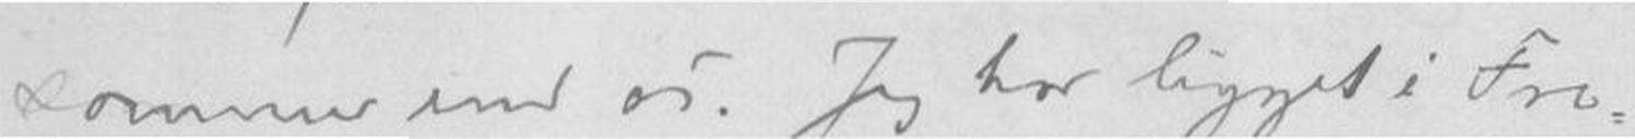sommer end os. Jeg har ligget i Fre-
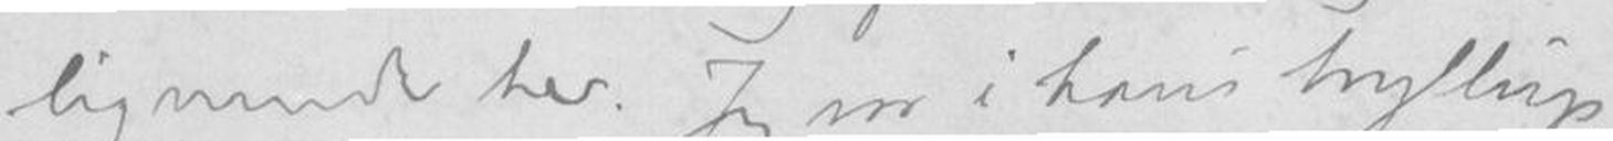lignende her. Jeg var i hans bryllup,
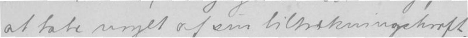at tabe noget af sin tiltrekningskraft
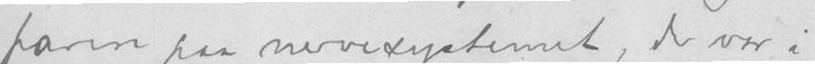parere paa nervesystemet, der var i
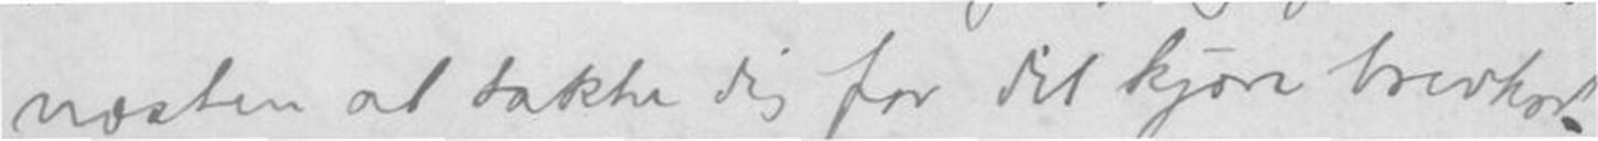næsten at takke dig for det kjøn brevkort
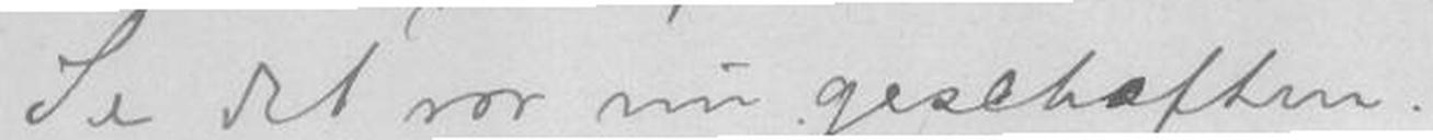Se det var min geschaften
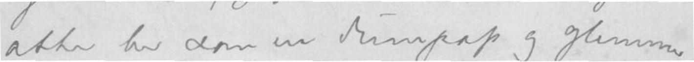atter her som en Dumpap og glemmer
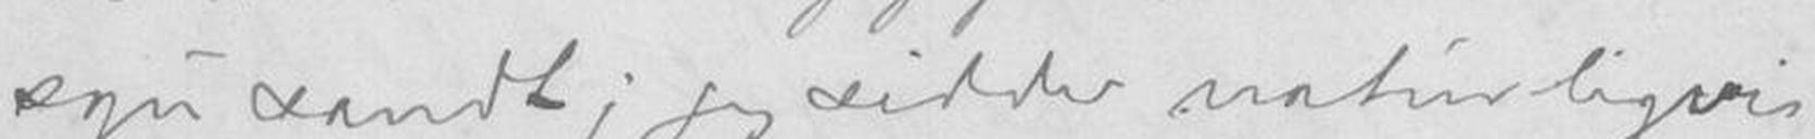sgu sandt, jeg sidder naturligvis
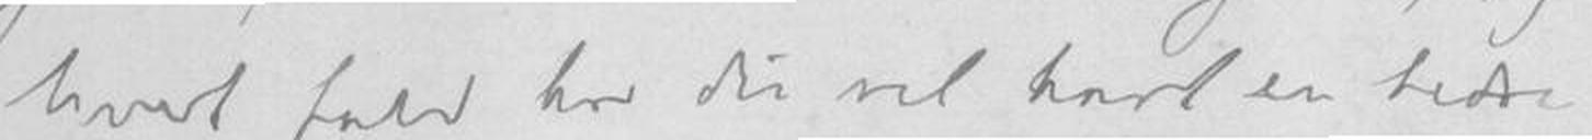hvert fald har du vel havt en bedre
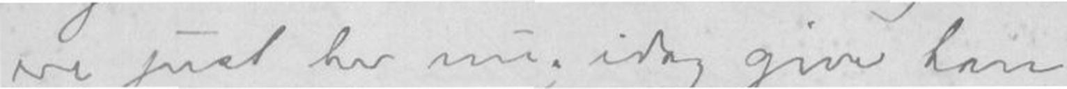ere just her nu; idag giver han
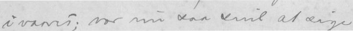i vaares, vær nu saa snil at sige
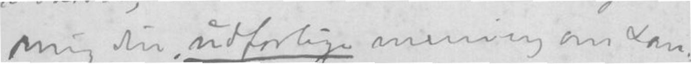mig din udforlige mening om san-
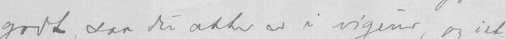godt, saa du atter er i vigeur, og iet-
